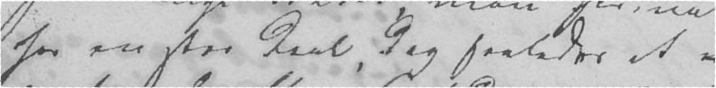for en stor Deel, dog saaledes at
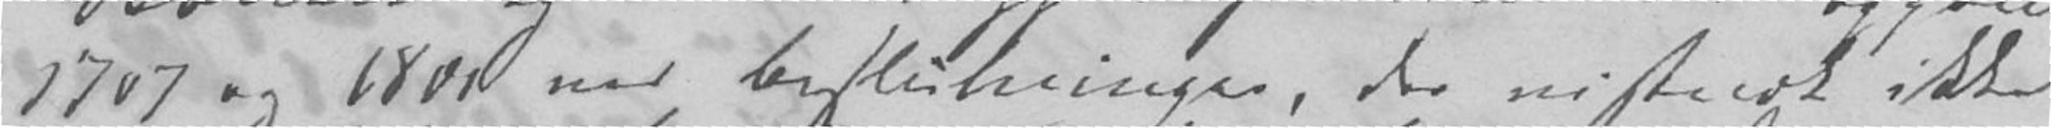1707 og 1808 ved Beslutninger, der vistnok ikke
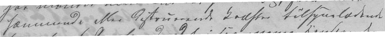hæmmende eller destruerende Kræfter tilsyneladende
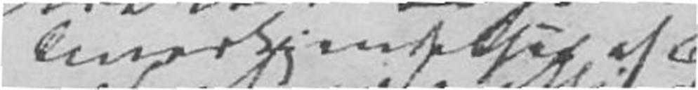Anerkjendelsen af
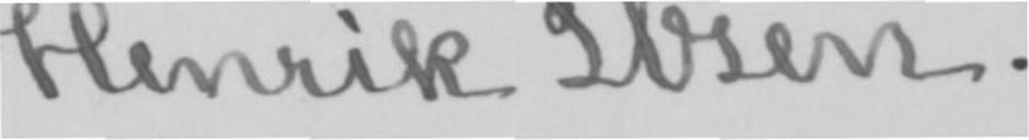Henrik Ibsen.
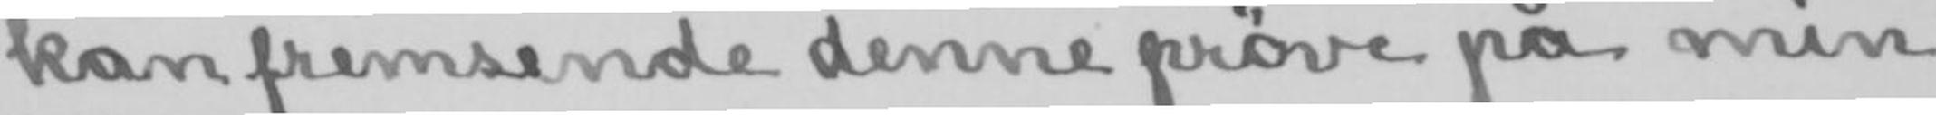kan fremsende denne prøve på min
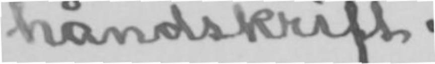håndskrift.
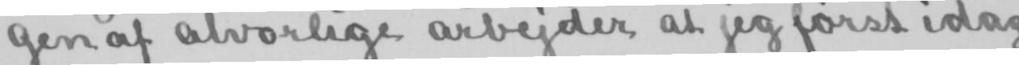gen af alvorlige arbejder at jeg først idag
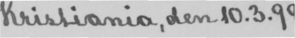Kristiania, den 10.3.99.
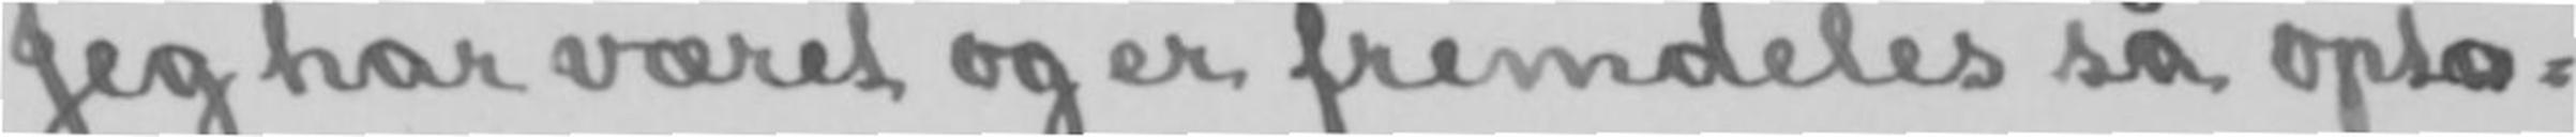Jeg har været og er fremdeles så opta-
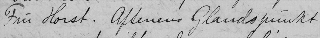Fru Horst. Aftenens Glandspunkt
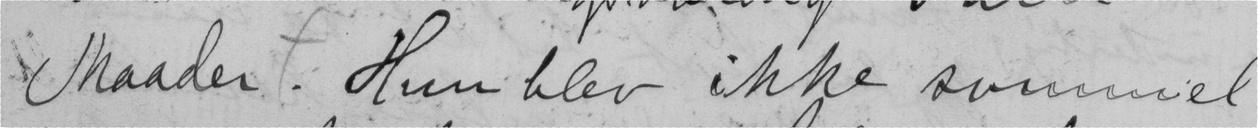Maader. Hun blev ikke svimmel
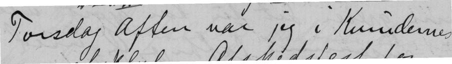Torsdag aften var jeg i Kvindernes
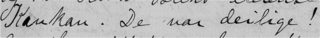Kankan. De var deilige!
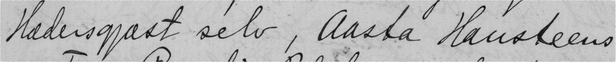Hædersgjæst selv, Aasta Hansteens
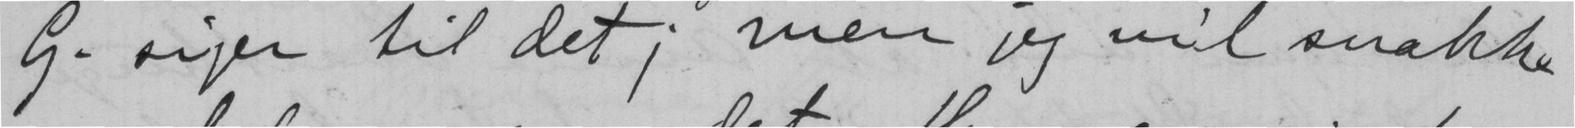G. siger til det; men jeg vil snakke
